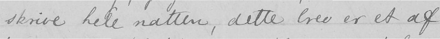skrive hele natten, dette brev er et af
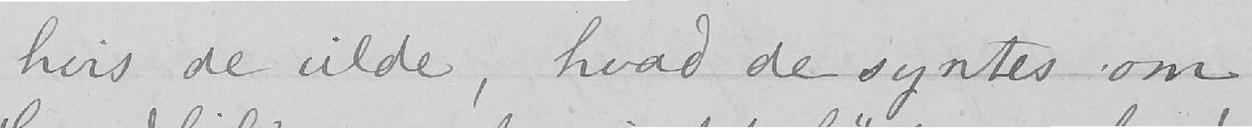hvis de vilde, hvad de syntes om
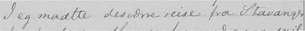Jeg maatte desværre reise fra Stavanger
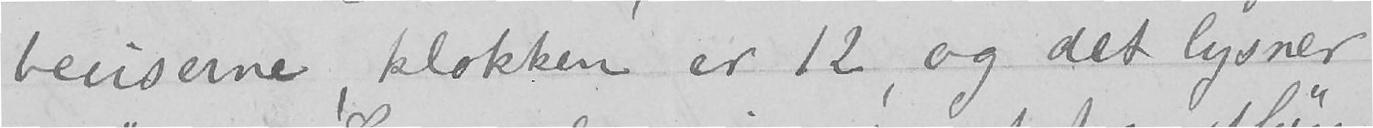beviserne, klokken er 12, og det lysner
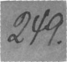249.
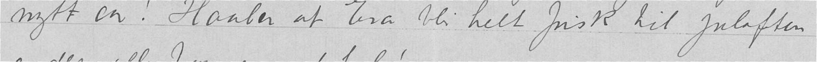nytt aar! Haaber at Eva bli helt frisk til julaften
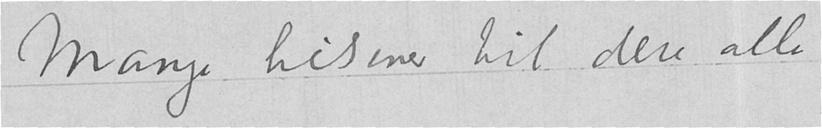Mange hilsener til dere alle
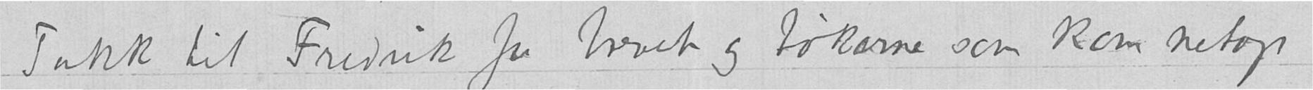Takk til Fredrik for brevet og bøkerne som kom netop
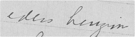eders hengivne
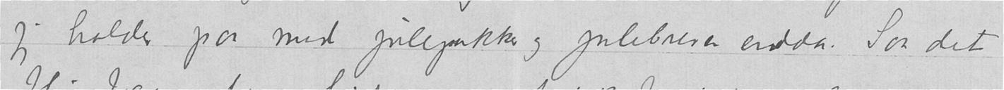jeg holder paa med julepakker og julebrever endda. Saa det
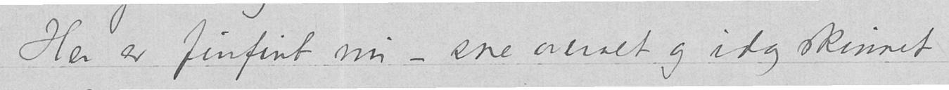Her er finfint nu – sne overalt og idag skinnet
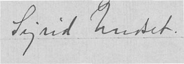Sigrid Undset.
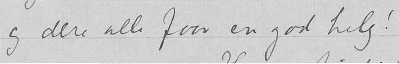og dere alle faar en god helg!
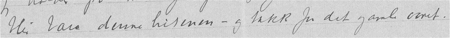blir bare denne hilsenen – og takk for det gamle aaret.
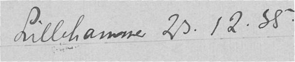Lillehammer 23.12.35.
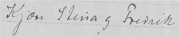Kjære Stina og Fredrik
